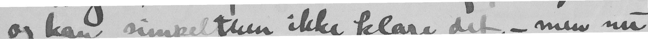og kan simpelthen ikke klare det, - men nu
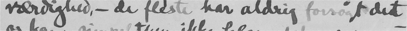værdighed, - de fleste har aldrig forsøgt det
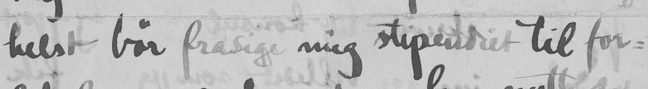helst bør frasige mig stipendiet til for-
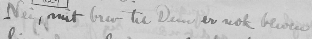Nei, mit brev til Dem er nok bleven
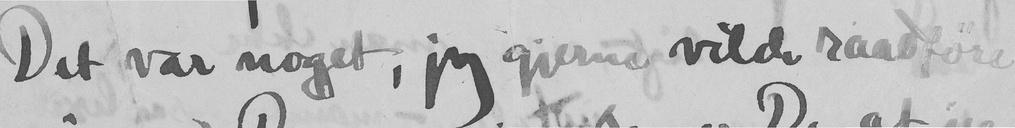Det var noget, jeg gjernes vilde raadføre
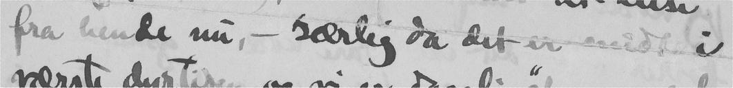fra hende nu, - særlig da det er midt i
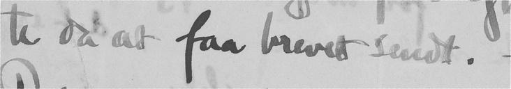te da at faa brevet sendt. -
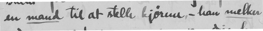en mand til at stelle kjørene, - han melker
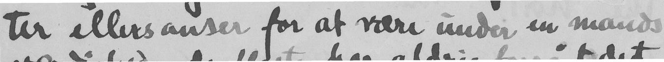ter ellers anser for at være under en mands
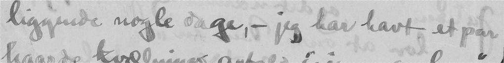liggende nogle dage, - jeg har havt et par
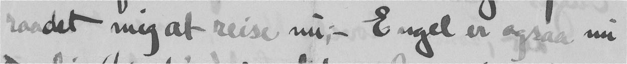raadet mig at reise nu : - Engel er ogsaa nu
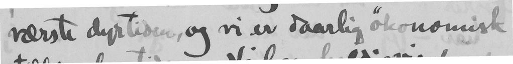værste dyrtiden, og vi er daarlig økonomisk
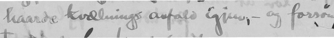haarde kvælnings anfal igjen, - og forsøm-
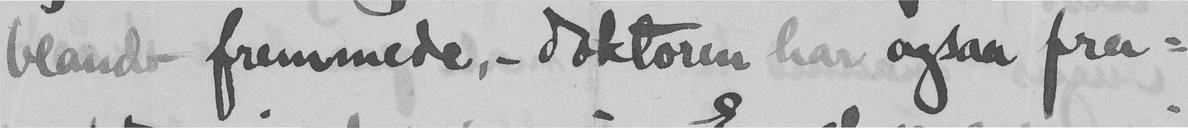blande fremmede, - doktoren har ogsaa fra-
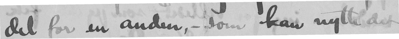del for en anden, - som kan nytte det
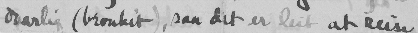daarlig (bronkit), saa det er leit at reise
